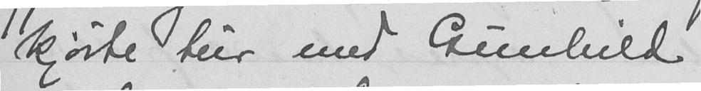kjørte tur med Gunhild
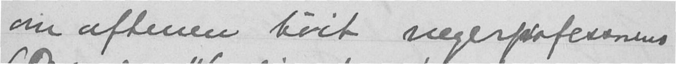om aftenen høit negerprofessorens
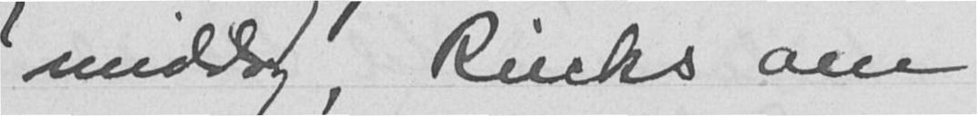middag, Riecks om
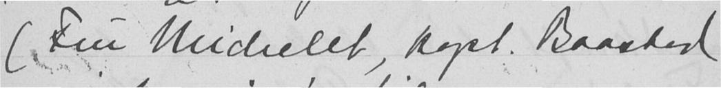(Fru Michelet, kapt. Baastad
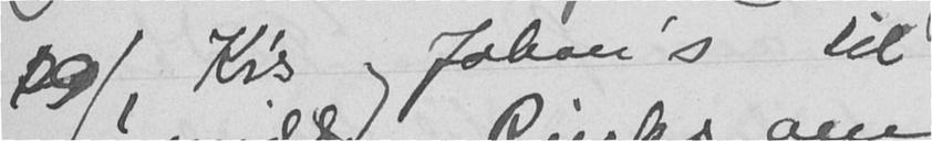19/1 Kr's og Johan's til
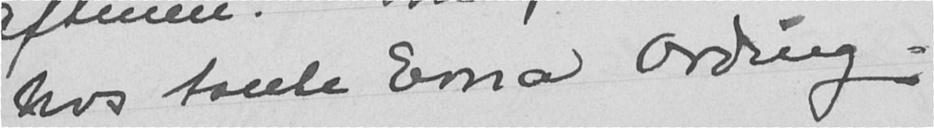hos tante Erna Ording.
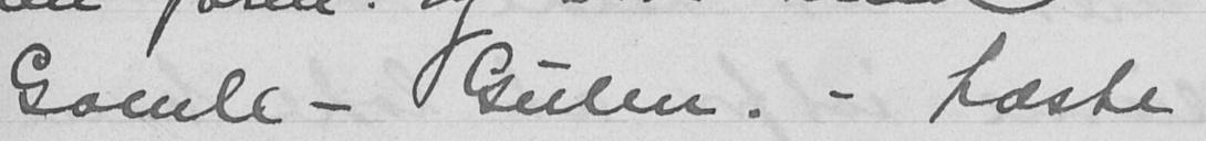Gamle-Gulen. - Læste
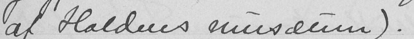af Haldens musæum).
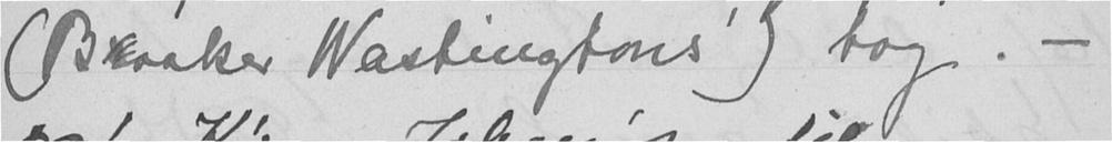(Blooker Washingtons') bog. -
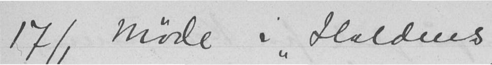17/1 Møde i "Haldens
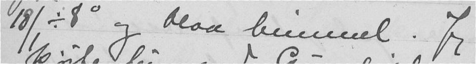18/1 ÷ 8º og blaa himmel. Jeg
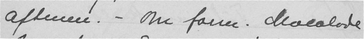aftenen. - Om form. chocolade
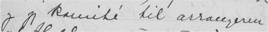og jeg komité til arrangeren
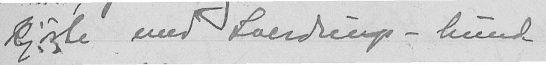kjørte med Sverdrup-hund
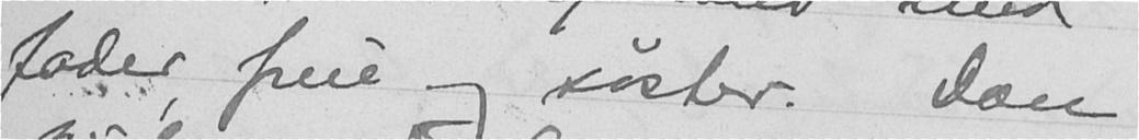fader frue og søster. Don
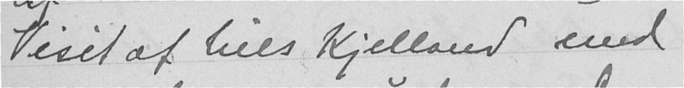Visit af Nils Kjelland med
